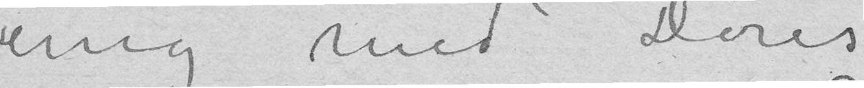enig med Deres
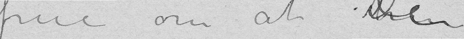frue om at De
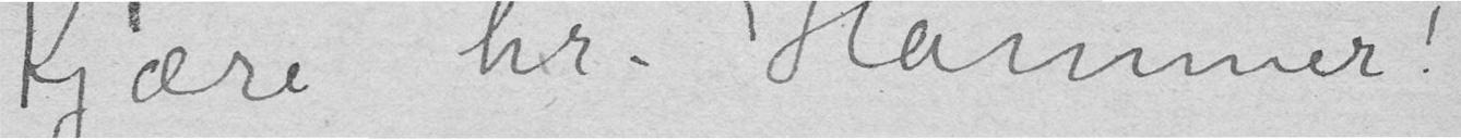Kjære hr. Hammer!
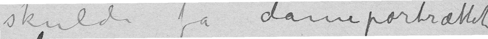skulde få dameportrættet
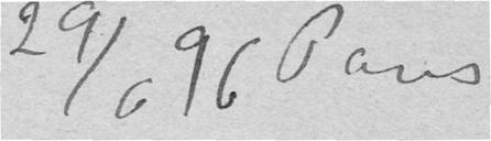29/6 96 Paris
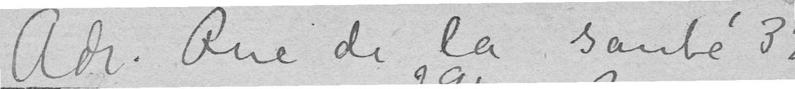Adr. Rue de la santé 32
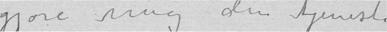gjøre mig den tjeneste
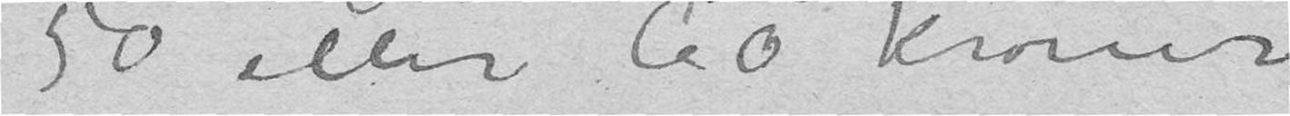50 eller 60 Kroner
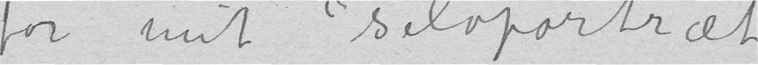for mit selvportræt –
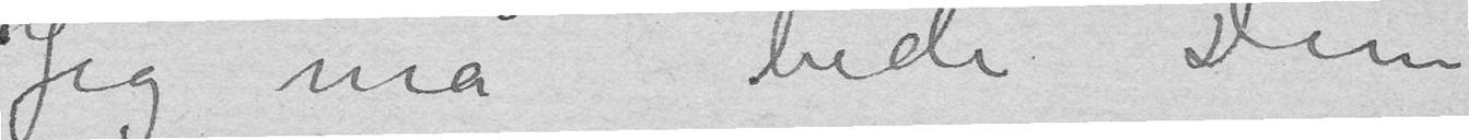Jeg må bede Dem
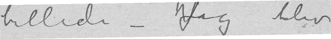billede – Jeg blev
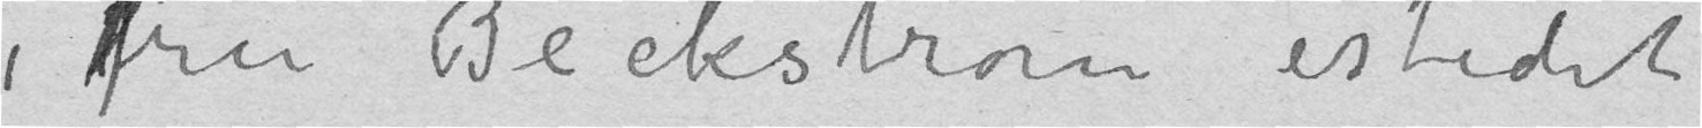Fru Beckström istedet
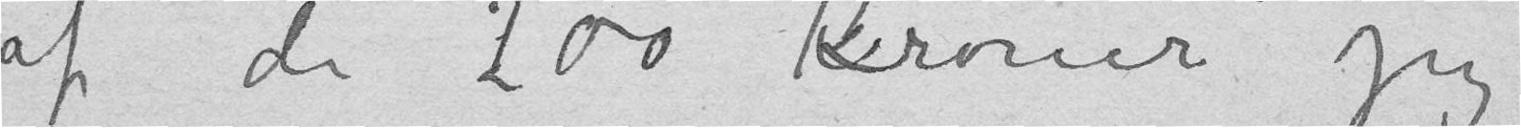af de 200 Kroner jeg
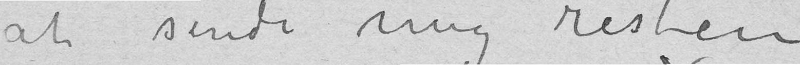at sende mig resten
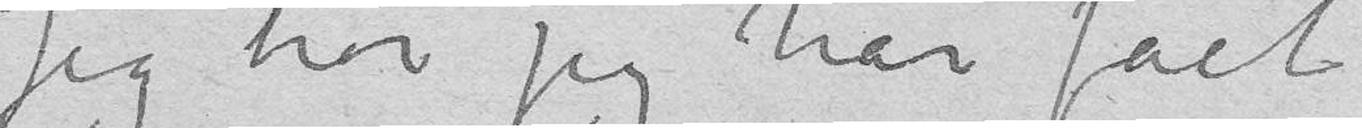Jeg tror jeg har fået
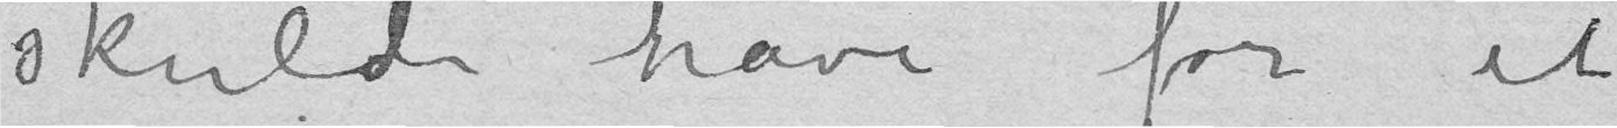skulde have for et
